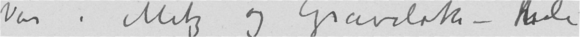Var i Metz, og Gravelotte – hele
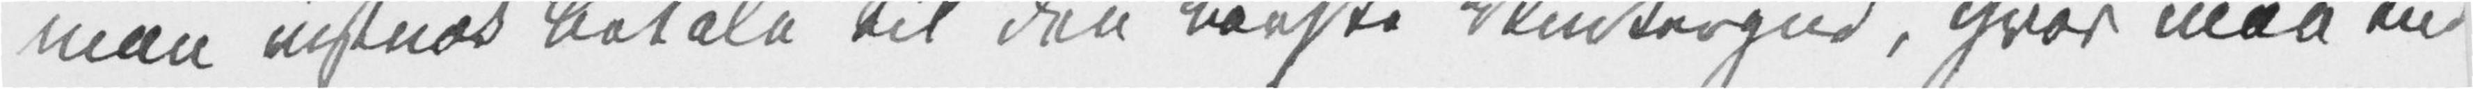man vistnok betale til den barske Vintergud, hvor man en[d]
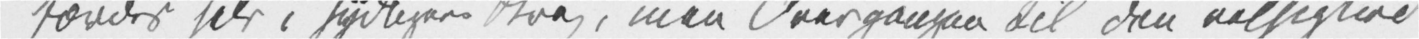færdes selv i sydligere Strøg, men Overgangen til den velsigned[e]
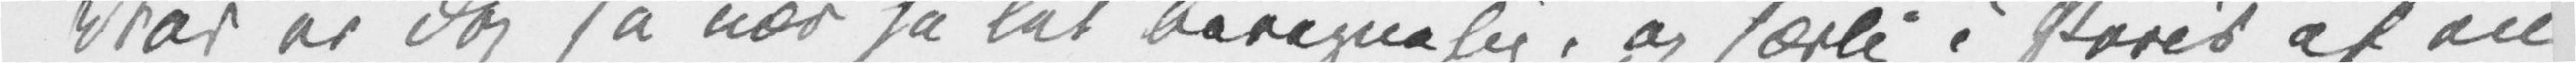Vår er dog så nær så let beregnelig, og særlig i Paris af en
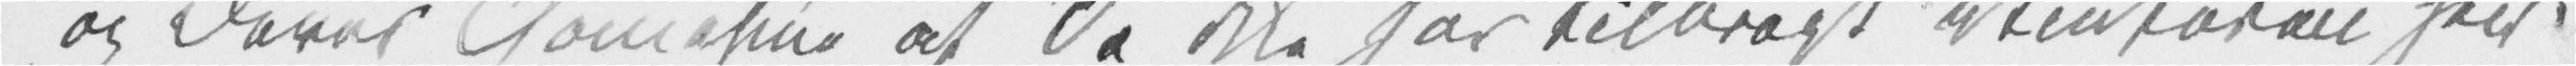og Deres Thomasine at De ikke har tilbragt Vinteren her[.]
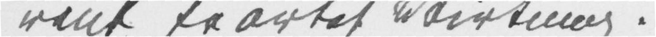rent feartet Virkning.
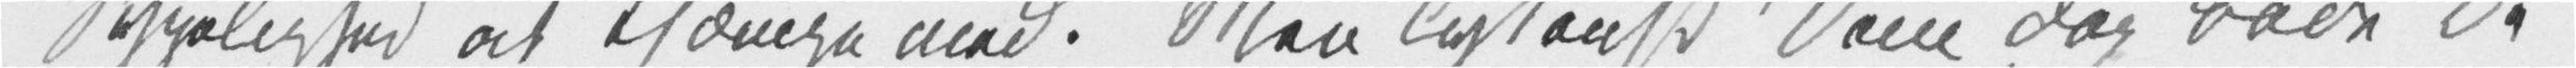Sygelighed at kjæmpe med. Men lykønsk Dem dog både De
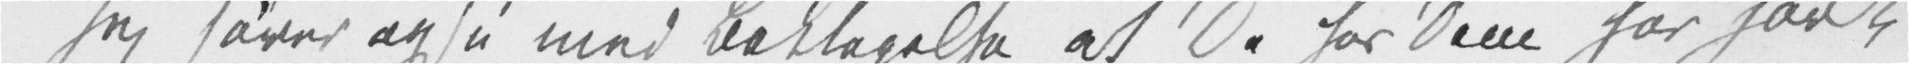Jeg hører også med Beklagelse at De hos Dem har havt
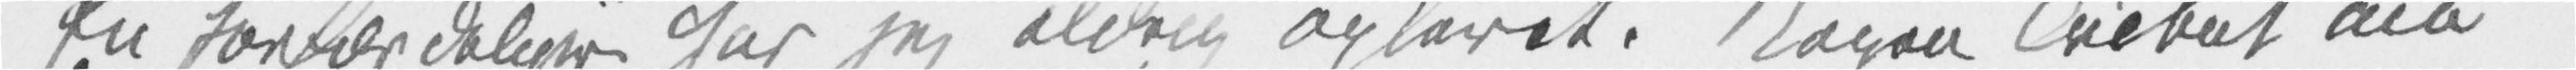En forfærdeligere har jeg aldrig oplevet. Nogen Tribut må
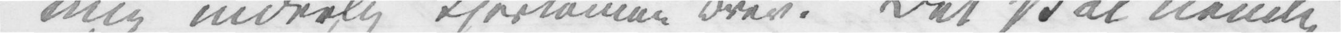mig inderlig kjerkomne Brev. Det skal nemlig
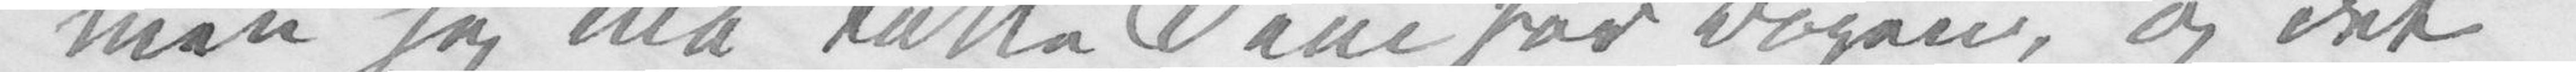men jeg må takke Dem for Bogen, og det
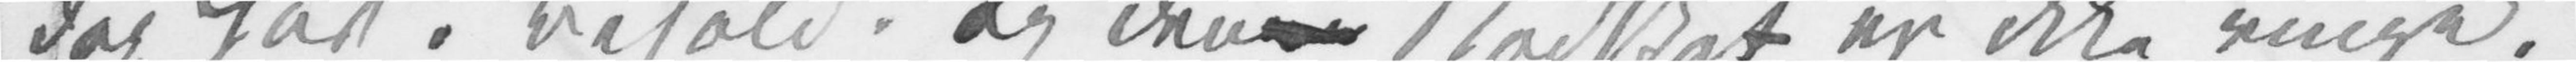dog har i Behold, og den ne Nødskat er ikke ringe.
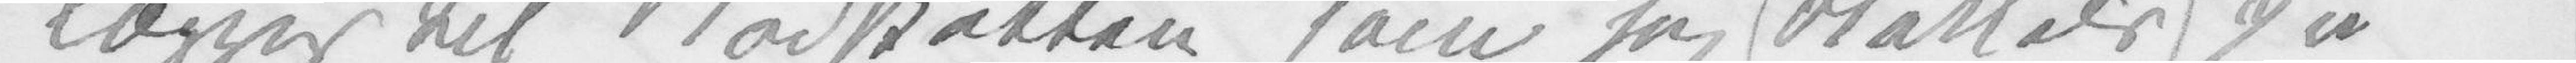lægges til «Nødskatten» som jeg Stakkels på
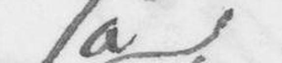så
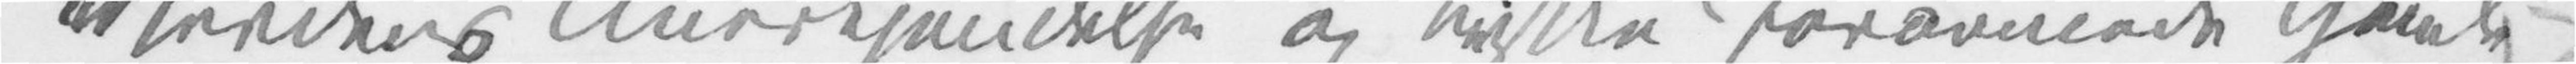Verdens Anerkjendelse og Lykke forarmede Gamle,
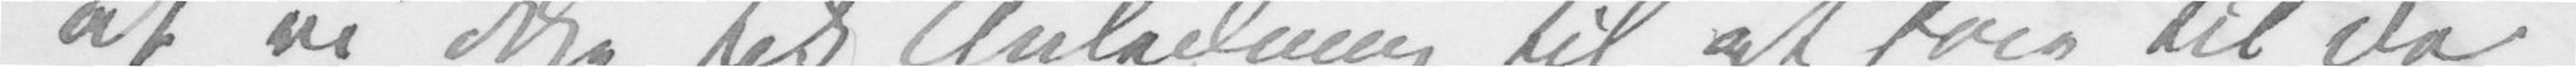at vi ikke fik Anledning til at føie til de
